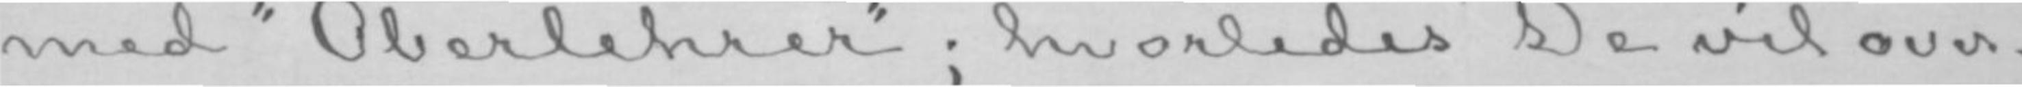med «Oberlehrer»; hvorledes De vil over-
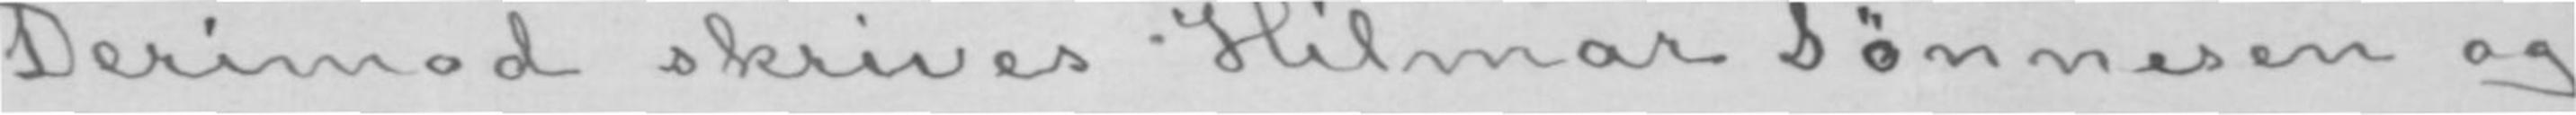Derimod skrives Hilmar Tønnesen og
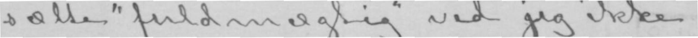sætte «fuldmægtig» ved jeg ikke.
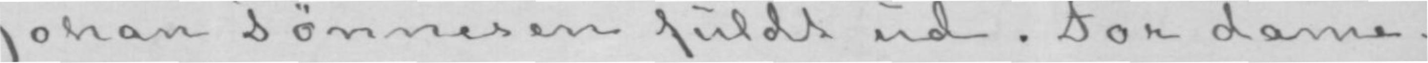Johan Tønnesen fuldt ud. For dame-
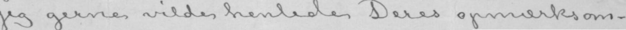jeg gerne vilde henlede Deres opmærksom-
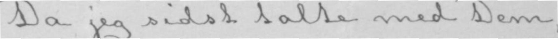Da jeg sidst talte med Dem,
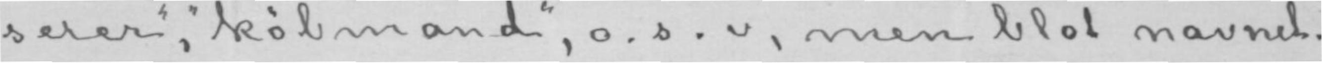serer», «købmand», o. s. v, men blot navnet.
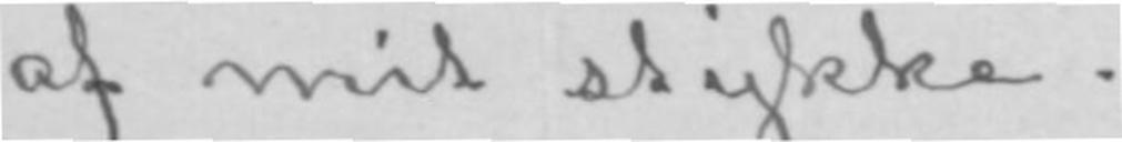af mit stykke.
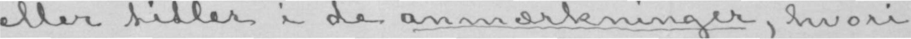eller titler i de anmærkninger, hvori
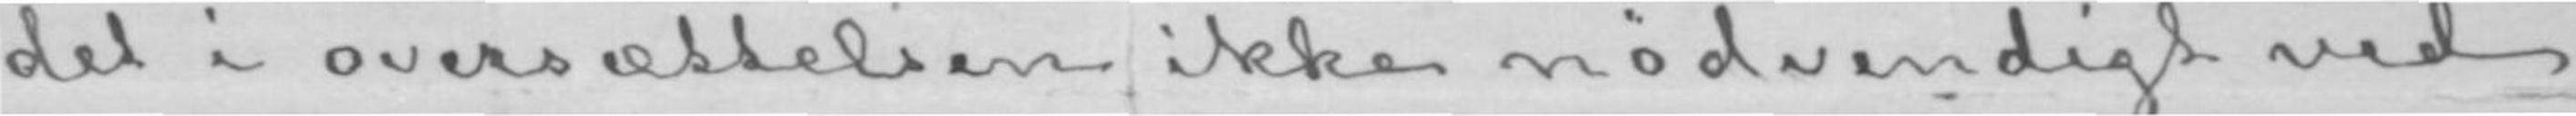det i oversættelsen ikke nødvendigt ved
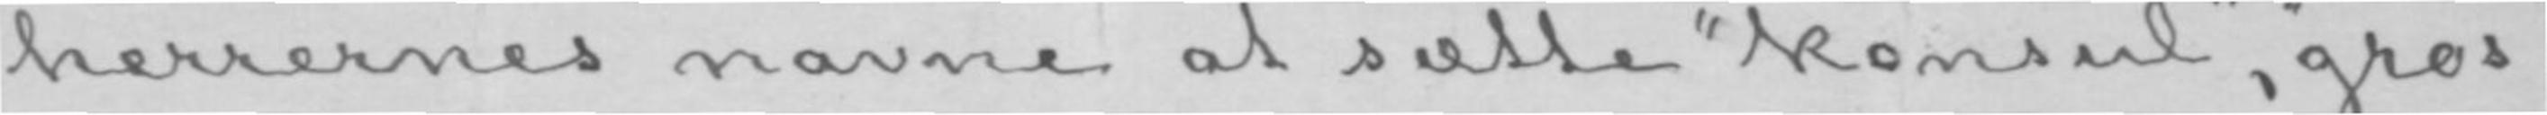herrernes navne at sætte «konsul», «gros-
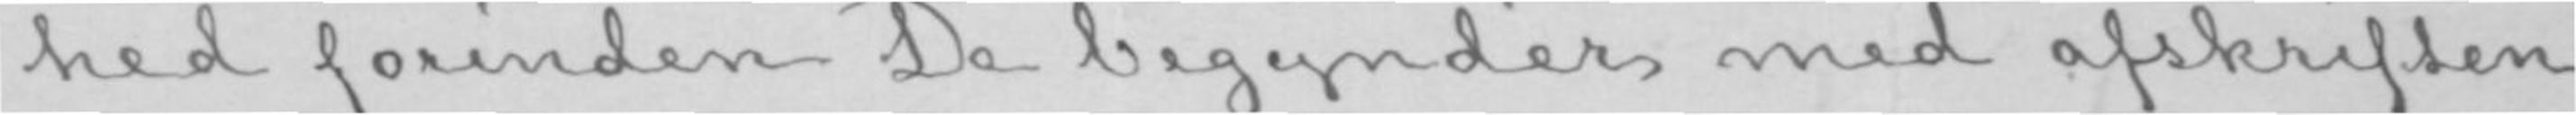hed forinden De begynder med afskriften
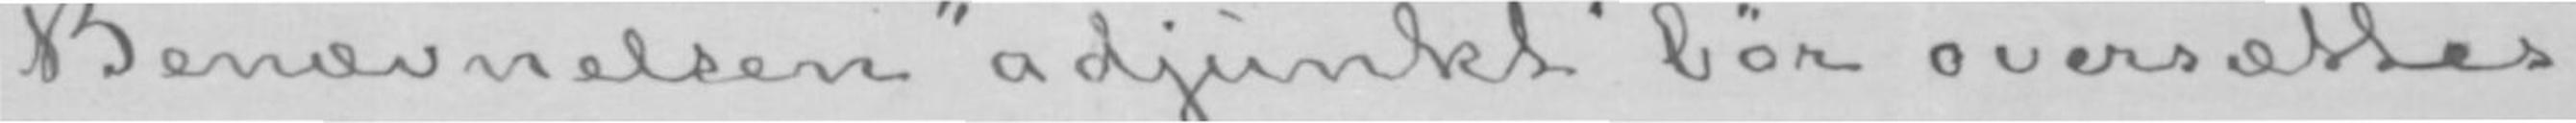Benævnelsen «adjunkt» bør oversættes
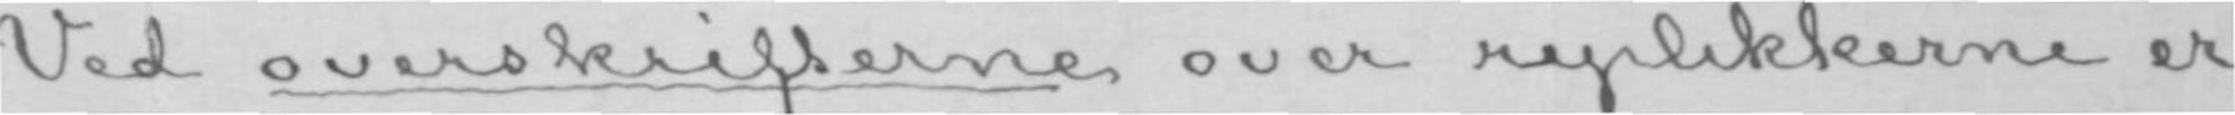Ved overskrifterne over replikkerne er
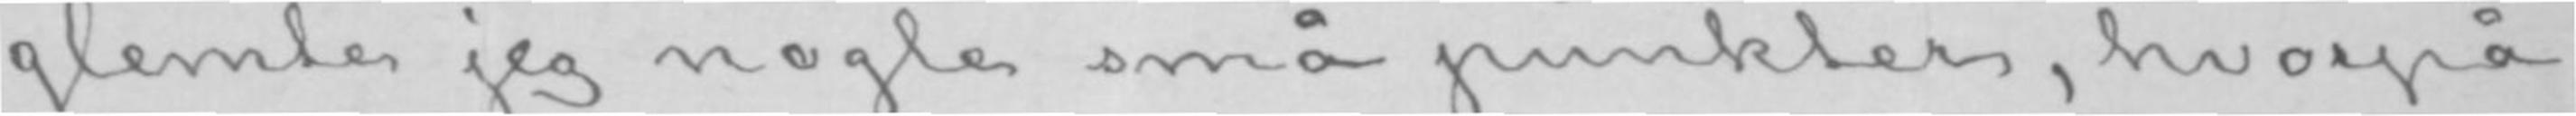glemte jeg nogle små punkter, hvorpå
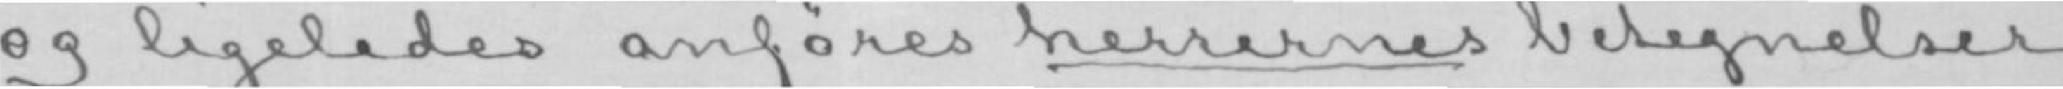og ligeledes anføres herrernes betegnelser
